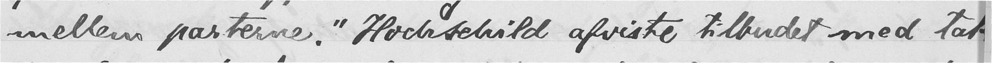mellem parterne." Hochschild afviste tilbudet med tak
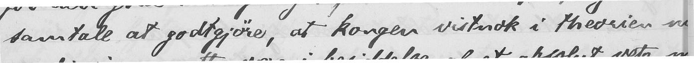samtale at godtgjøre, at kongen vistnok i theorien nød-
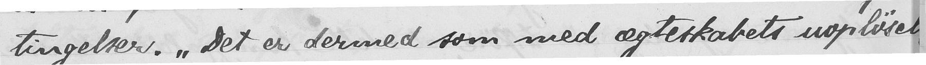tingelser. "Det er dermed som med ægteskabets uopløselig-
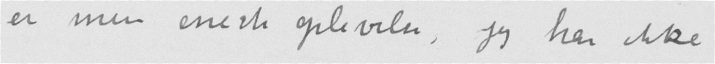er min eneste oplevelse, jeg har ikke
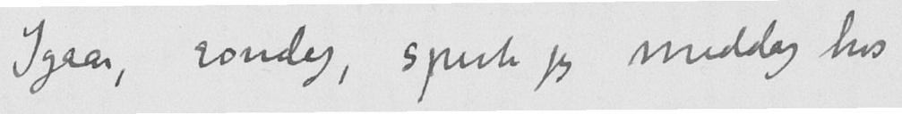Igaar, søndag, spiste jeg middag hos
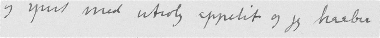og spist med utrolig appetit og jeg haaber
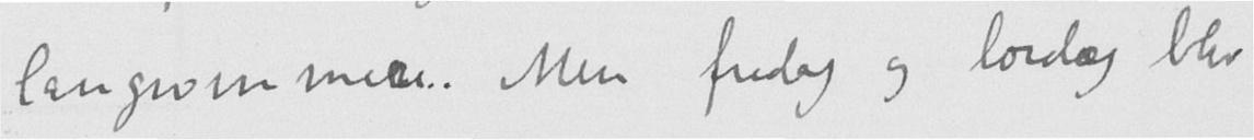langsommere. Men fredag og lørdag blev
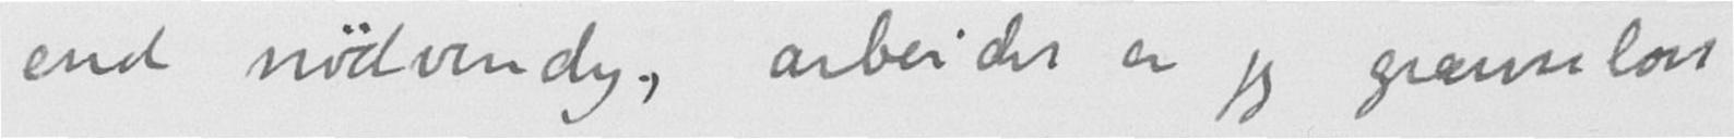end nødvendig, arbeidet er jeg grænseløst
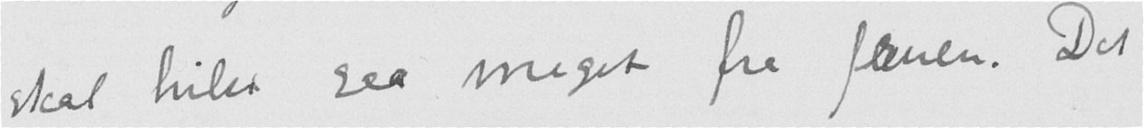skal hilse saa meget fra fruen. Det
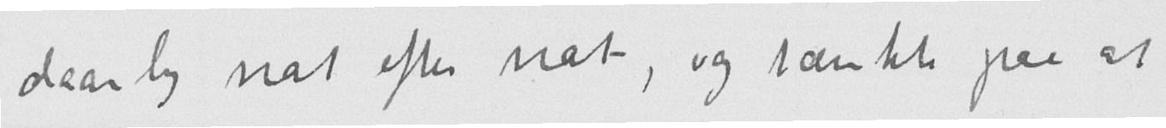daarlig nat efter nat, og tænkte paa at
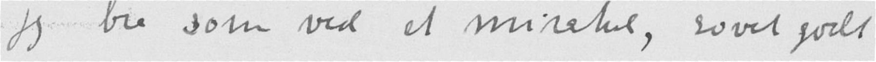jeg bra som ved et mirakel, sovet godt
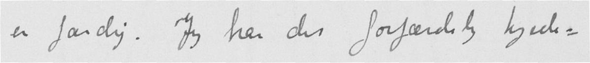er færdig. Jeg har det forfærdelig kjede-
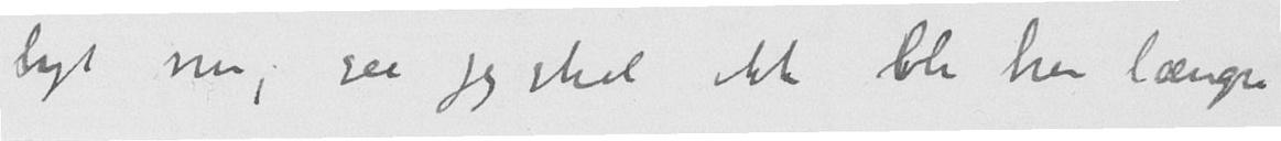ligt nu, saa jeg skal ikke bli her længre
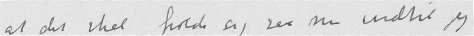at det skal holde sig saa nu indtil jeg
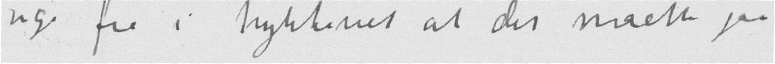sige fra i trykkeriet at det maatte gaa
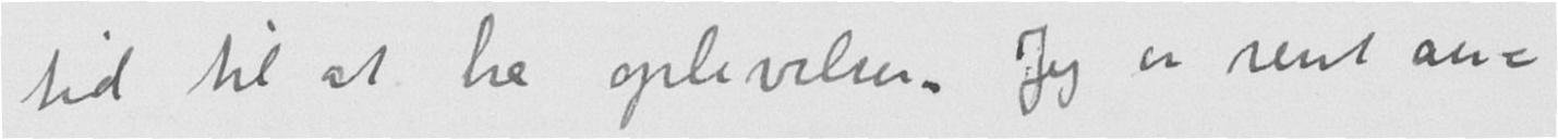tid til at ha oplevelser. Jeg er rent au-
